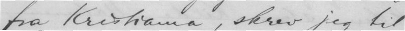fra Kristiania, skrev jeg til
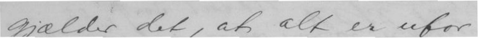gjælder det, at alt er ufor-
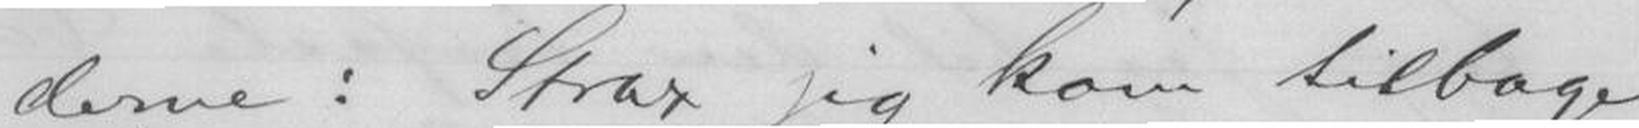derne: Strax jeg kom tilbage
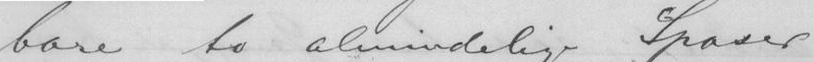bare to almindelige Spaser-
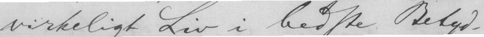virkeligt Liv i bedste Betyd-
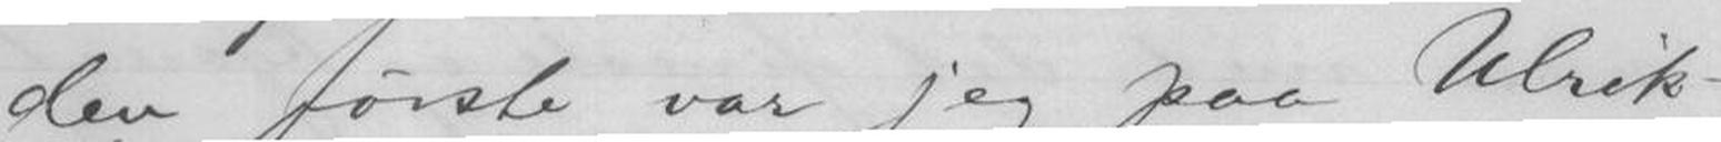den første var jeg paa Ulrik-
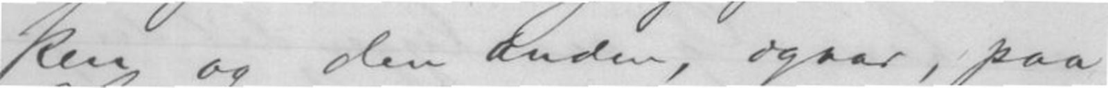ken og den anden, igaar, paa
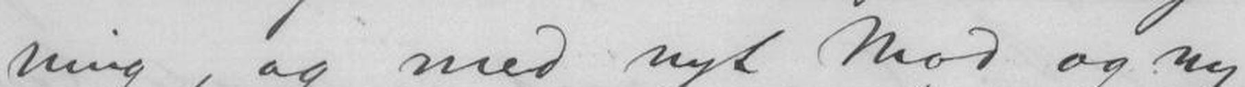ning, og med nyt Mod og ny
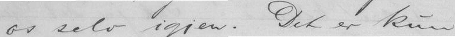os selv igjen. Det er kun
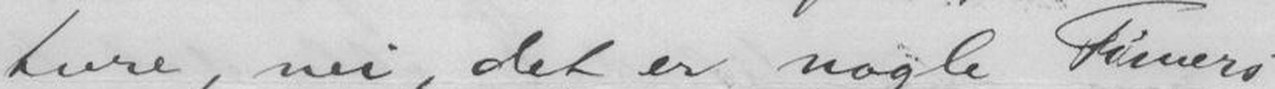ture, nei, det er nogle Timers
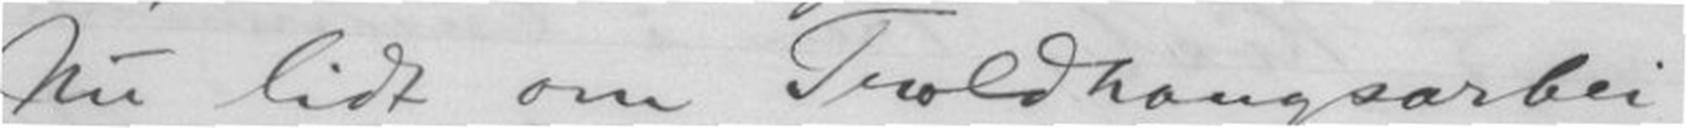Nu lidt om Troldhaugsarbei-
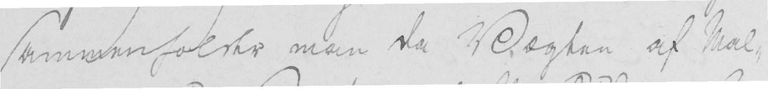sammenholder man da Vægten af Mal-
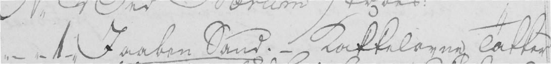"- "-1-" I aaben Sand. - Kakkelovne Takker
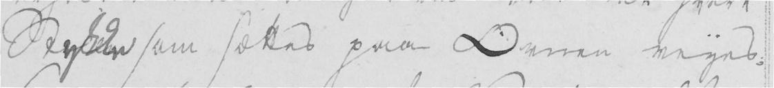Stykke som sættes paa Ovnen veyes;
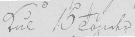Kul 15 Tønder
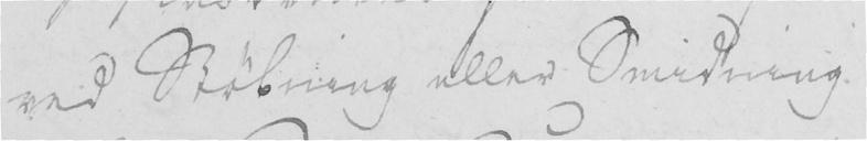ved Støbning eller Smidning
